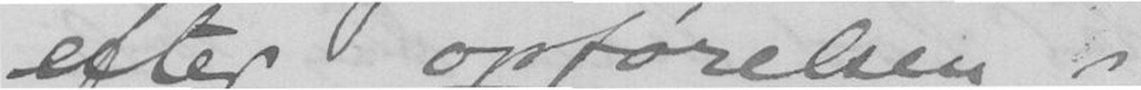efter opførelsen i
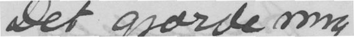Det gjorde mig
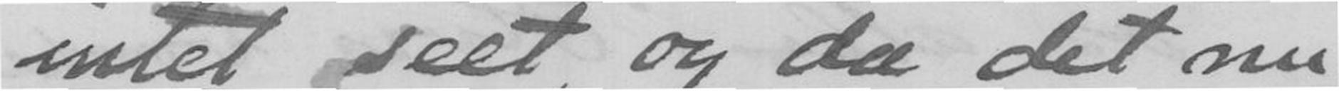intet seet, og da det nu
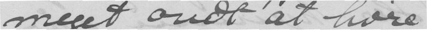meget ondt at høre
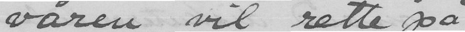våren vil rette på
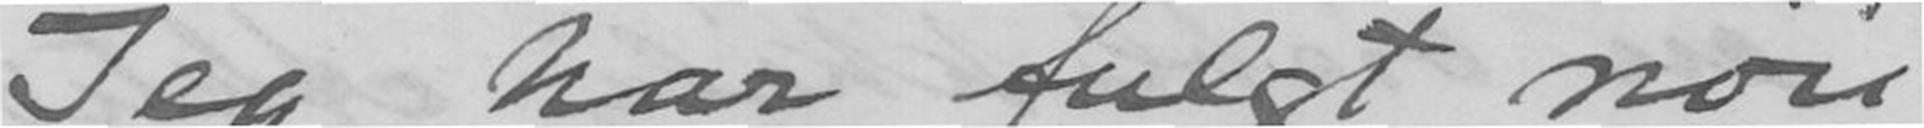Jeg har fulgt nøie
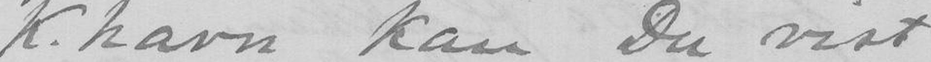K.havn kan Du vist
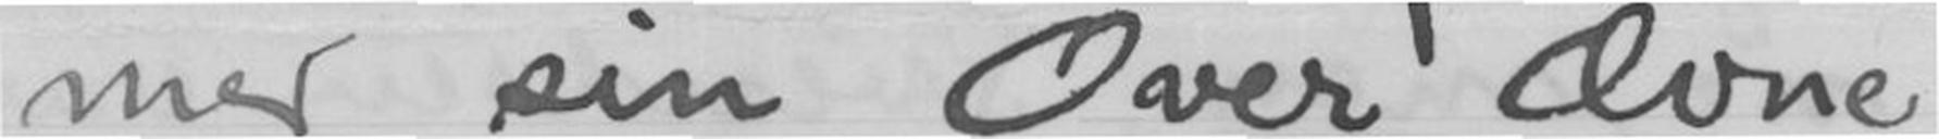med sin Over Ævne
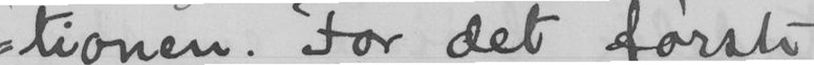tionen. For det første
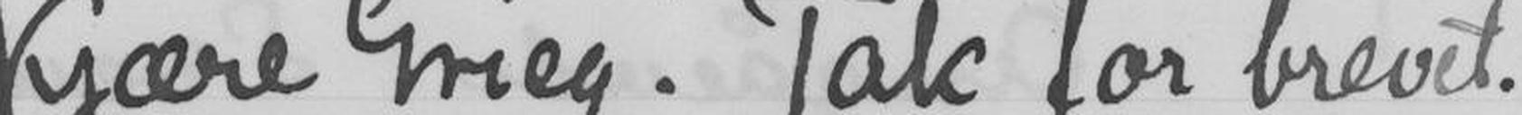Kjære Grieg! Tak for brevet.
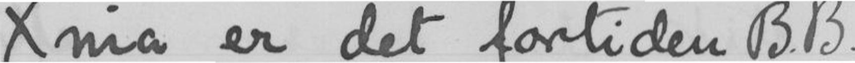Xnia er det fortiden B.B.
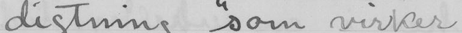digtning som virker
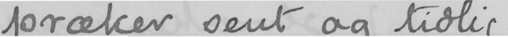præker sent og tidlig.
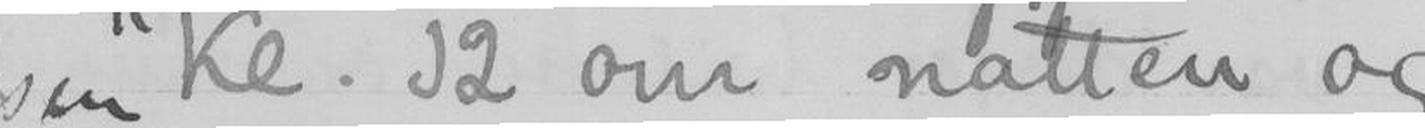en Kl. 12 om natten og
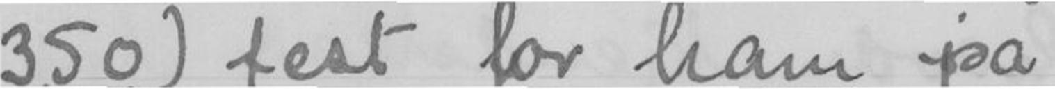350) fest for ham på
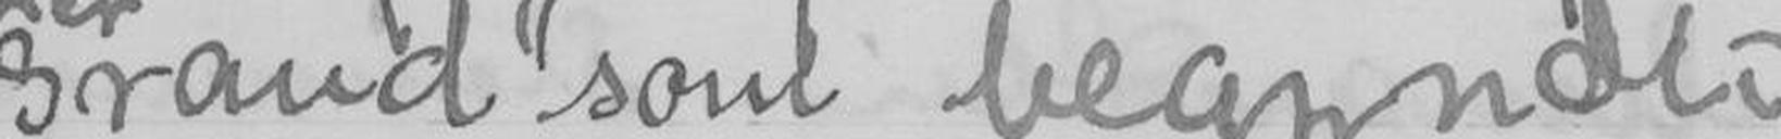Grand" som begyndte
